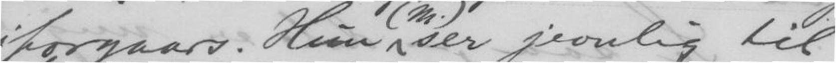iforgaars. Hun ser jevnlig til
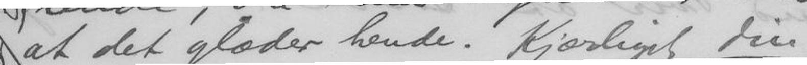at det glæder hende. Kjærligst Din
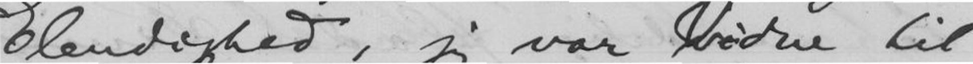Elendighed, jeg var Vidne til
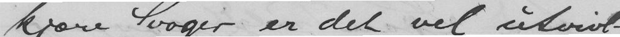kjære Svoger er det vel utvivl-
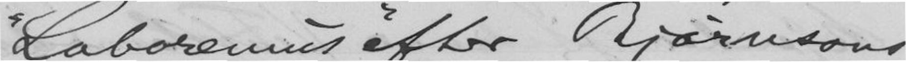"Laboremus" efter Bjørnsons
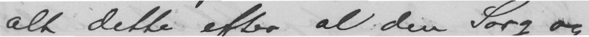alt dette efter al den Sorg og
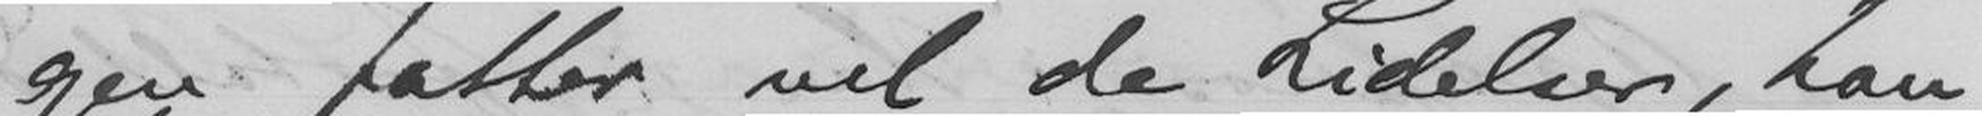gen fatter vel de Lidelser, han
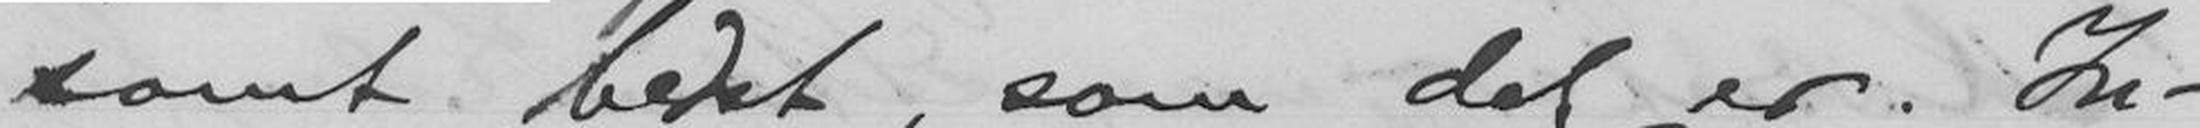somt bedst, som det er. In-
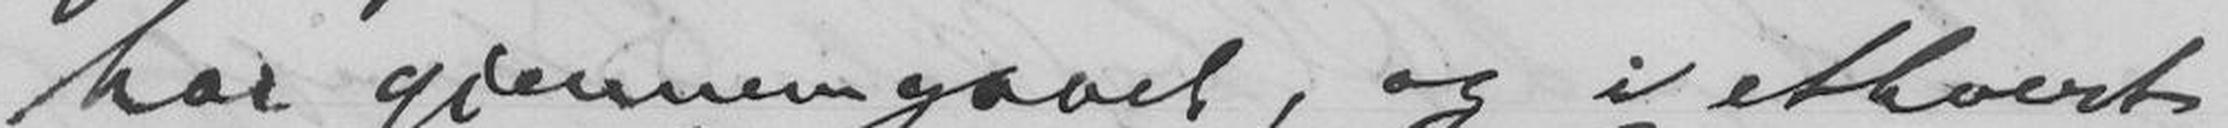har gjennemgaaet, og i ethvert
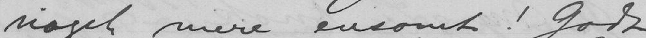noget mere ensomt! Godt
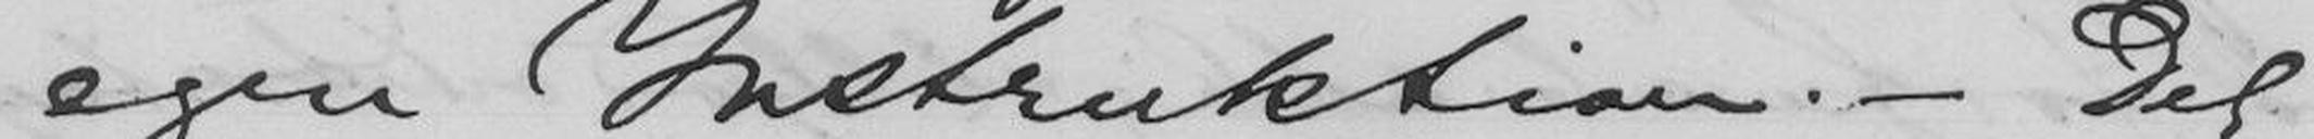egen Instruktion. - Det
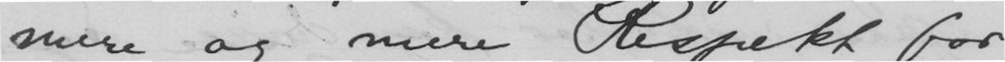mere og mere Respekt for
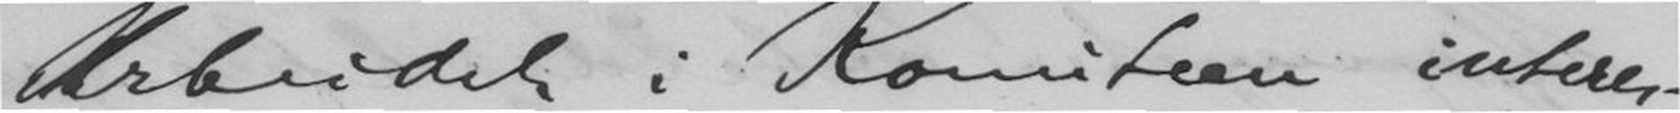Arbeidet i Komiteen interes-
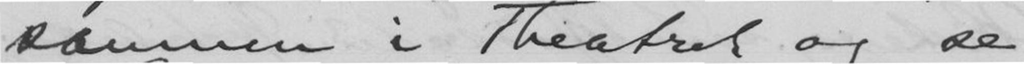sammen i Theatret og se
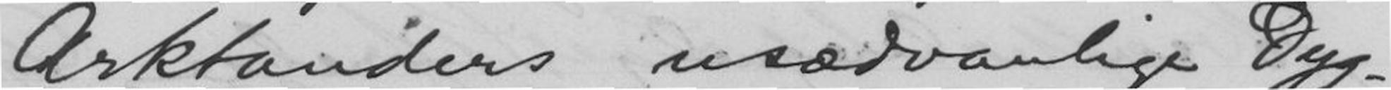Arktanders usædvanlige Dyg-
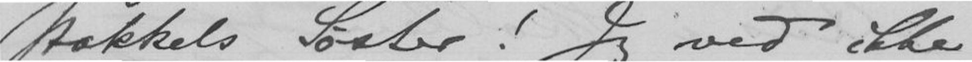stakkels Søster! Jeg ved ikke
